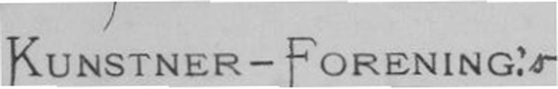KUNSTNER-FORENING:s
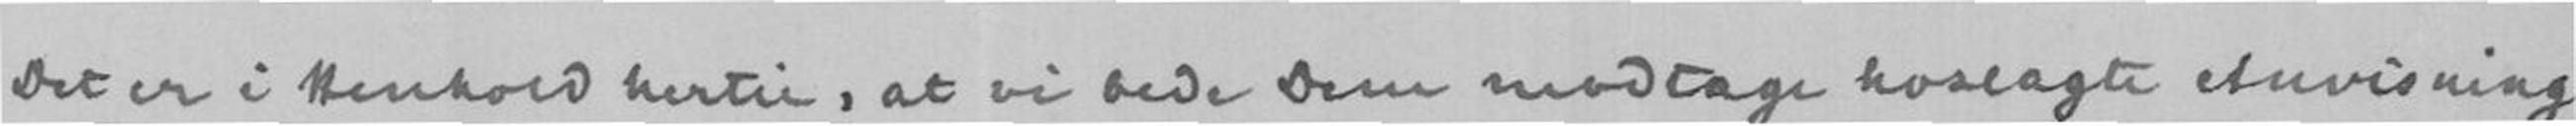Det er i Henhold hertil, at vi bede Dem modtage hoslagte Anvisning
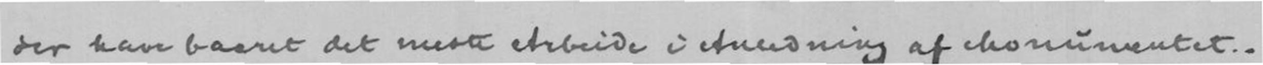der have baaret det meste Arbeide i Anledning af Monumentet.
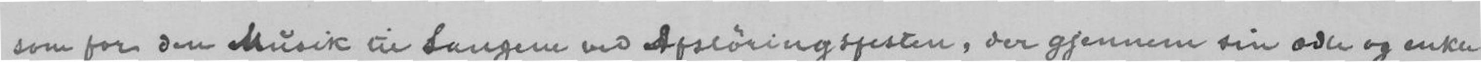for den Musik til Sangene ved Afsløringsfesten, den gjennem sin ædle og enkle
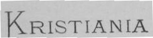KRISTIANIA
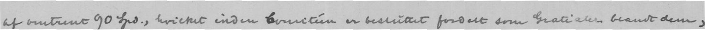af omtrent 90 Spd., hvilket inden Comitéen er besluttet fordelt som Gratialer blandt dem,
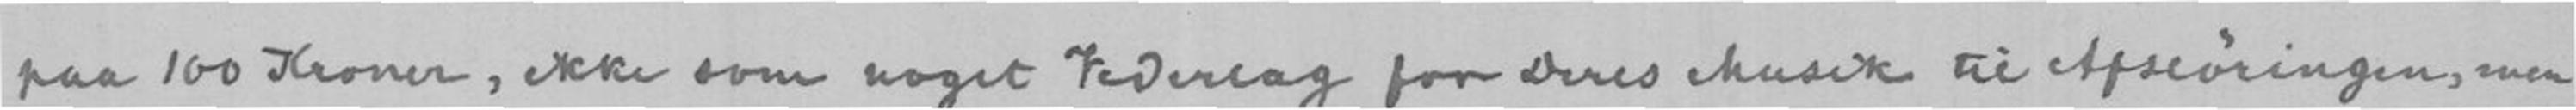paa 100 Kroner, ikke som noget Vederlag for Deres Musik til Afsløringen, men
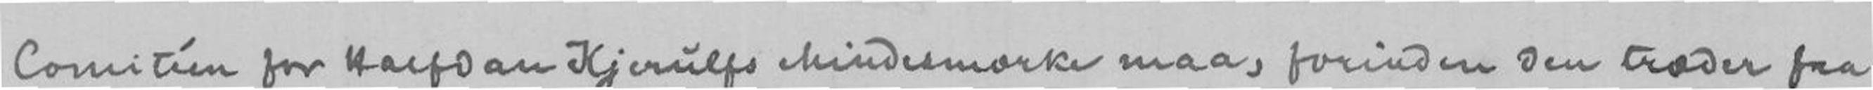Comitéen for Halfdan Kjerulfs Mindesmærke maa, forinden den træder fra
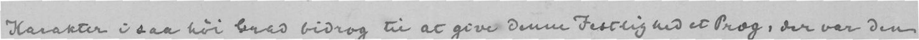Karakter i saa høi Grad bidrog til at give denne Festlighed et Præg, der var den
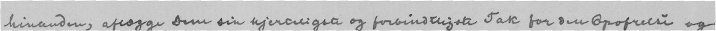hinanden, aflægge Dem sin hjerteligste og forbindtligste Tak for den Opofrelse og
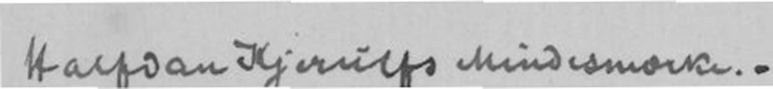Halfdan Kjerulfs Mindesmærke.
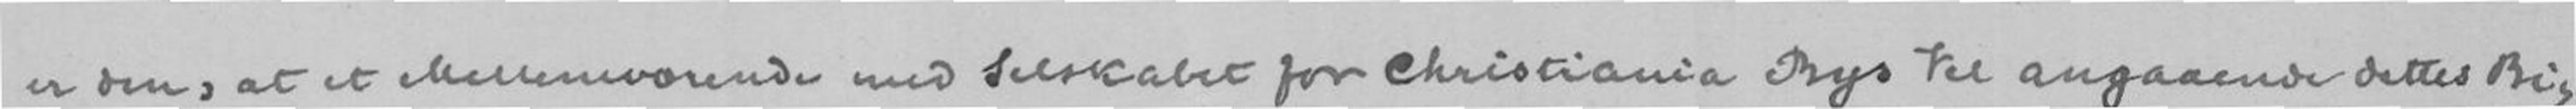er den, at et Mellemværende med Selskabet for Christiania Bys Vel angaaende dettes Bi-
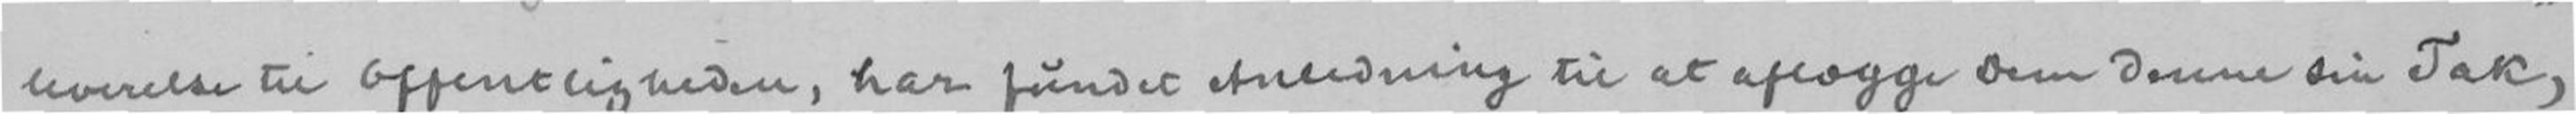leverelse til Offentligheden, har fundet Anledning til at aflægge Dem denne sin Tak,
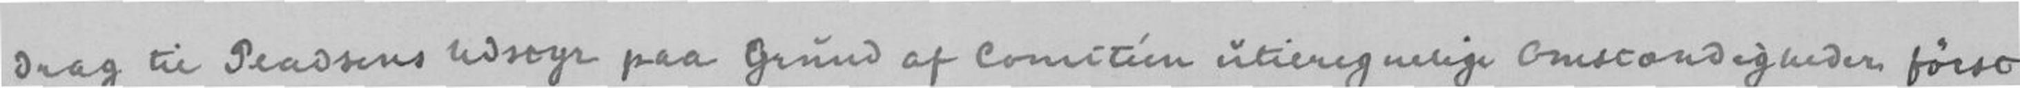drag til Pladsens Udstyr paa Grund af Comitéen utilregnelige Omstændigheder først
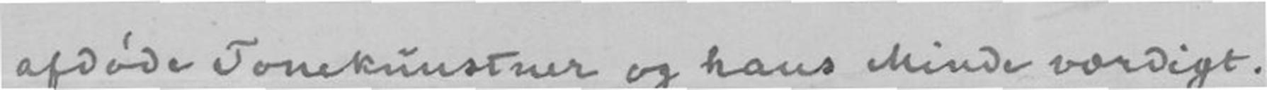afdøde Tonekunstner og hans Minde værdigt.
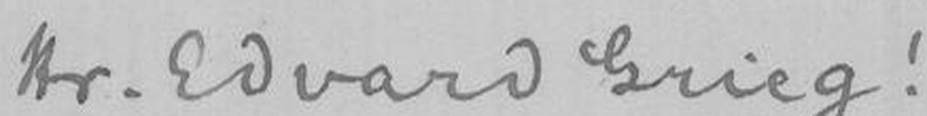Hr. Edvard Grieg!
